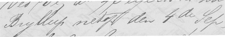Bryllup netop den 4de Sep-
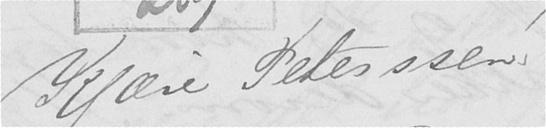Kjære Peterssen!
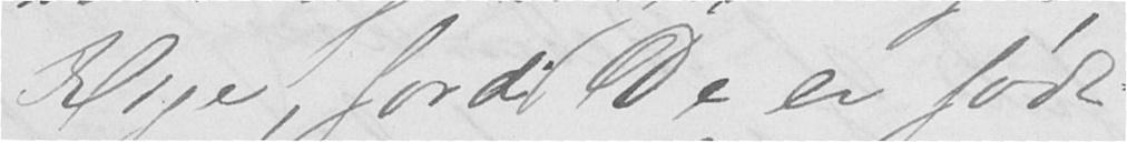Rige, fordi De er født.
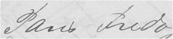Paris Fredag.
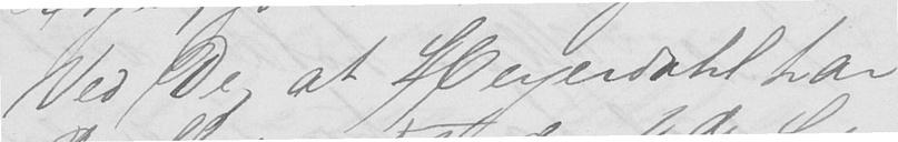Ved De, at Heyerdahl har
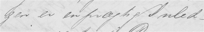gen er en prægtig Anled-
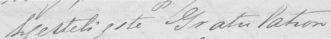hjerteligste Gratulation
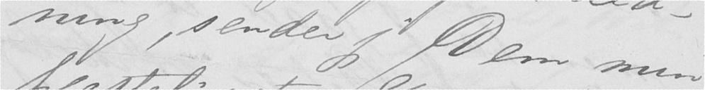ning, sender jeg Dem min
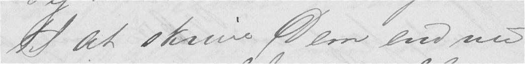til at skrive Dem endnu
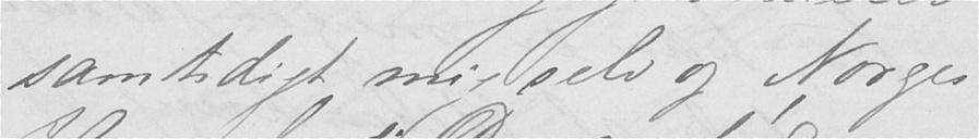samtidigt mig selv og Norges
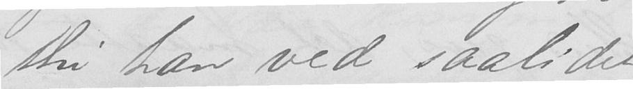thi han ved saalidet
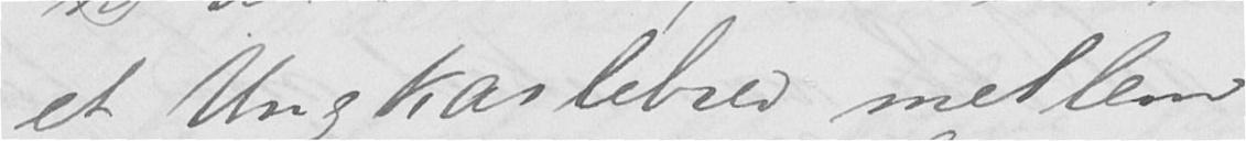et Ungkarlebrev mellem
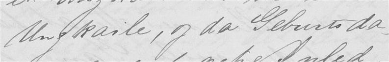Ungkarle, og da Geburtsda-
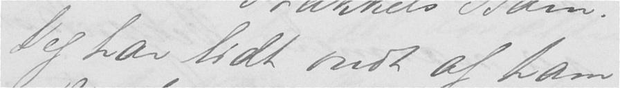Jeg har lidt ondt af ham,
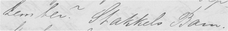tember? Stakkels Barn!
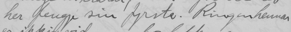her fengje sin fyrste. Ringen hennar
